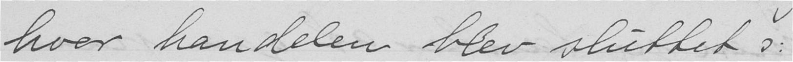hvor handelen blev sluttet:
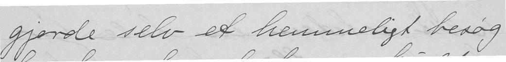gjorde selv et hemmeligt besøg
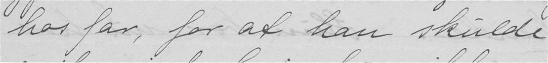hos far, for at han skulde
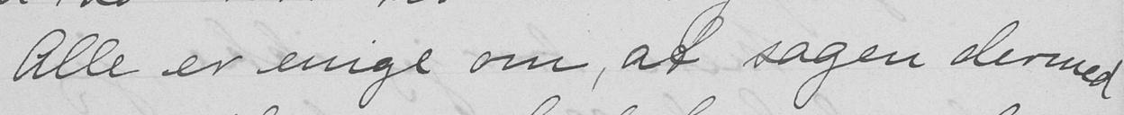Alle er enige om, at sagen dermed
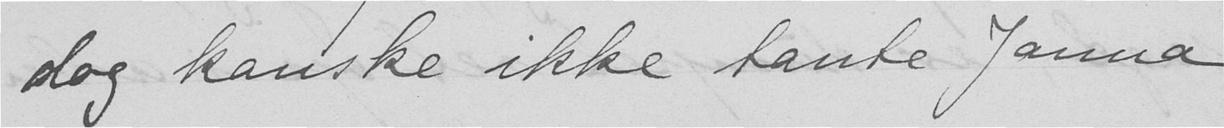dog kanske ikke tante Janna
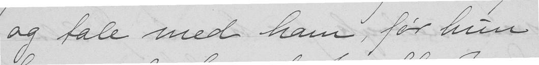og tale med ham, før hun
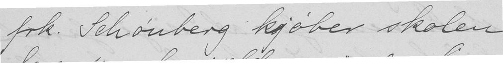frk. Schønberg kjøber skolen
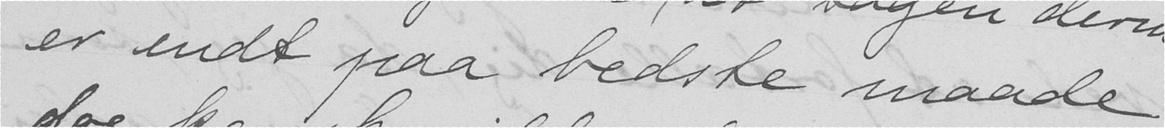er endt paa bedste maade;
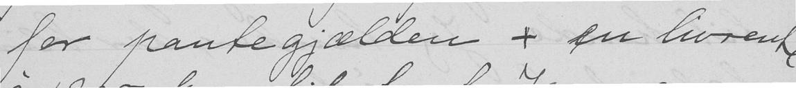for pantegjælden + en livrente
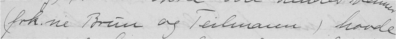(frk.ne Brun og Teilmann) havde
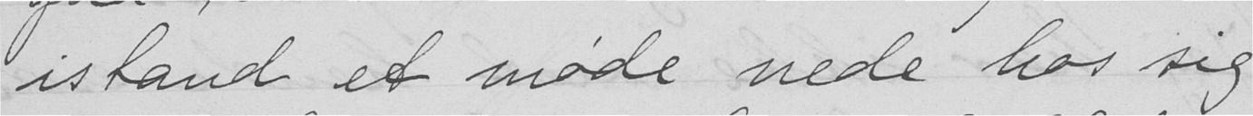istand et møde nede hos sig,
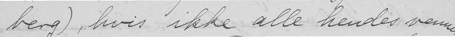berg), hvis ikke alle hendes venner
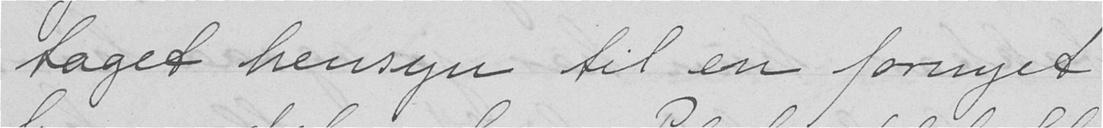taget hensyn til en fornyet
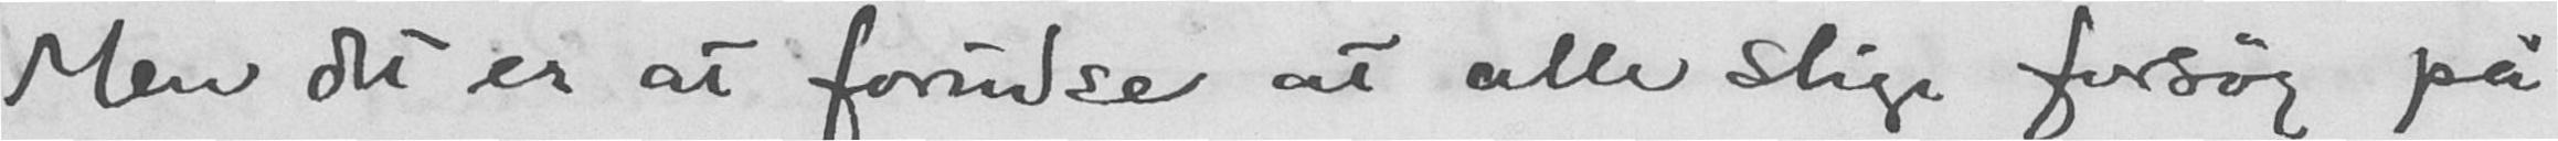Men det er at forudse at alle slige forsøg på
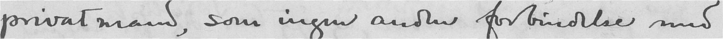privatmand, som ingen anden forbindelse med
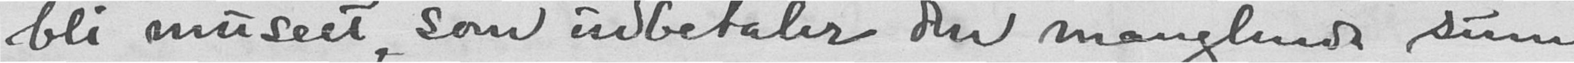bli musseet, som udbetaler den manglende sum
